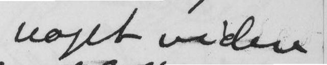noget videre
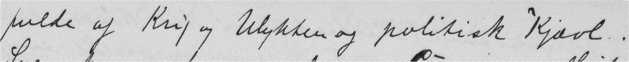fulde af Krig og Ulykten og politisk Kjævl.
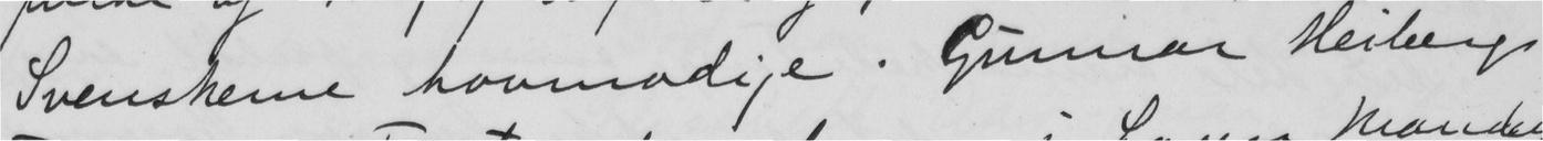Svenskerne hovmodige. Gunnar Heibergs
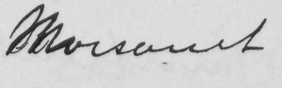Morsomt
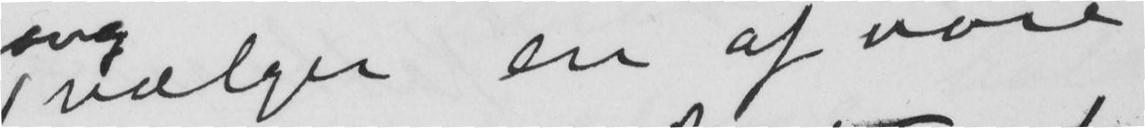vælger en af vore
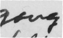gang
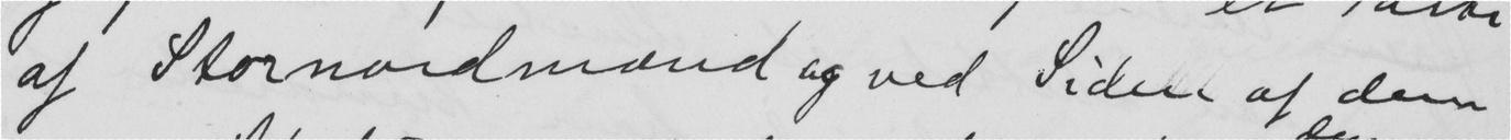af Stornordmænd og ved Siden af dem
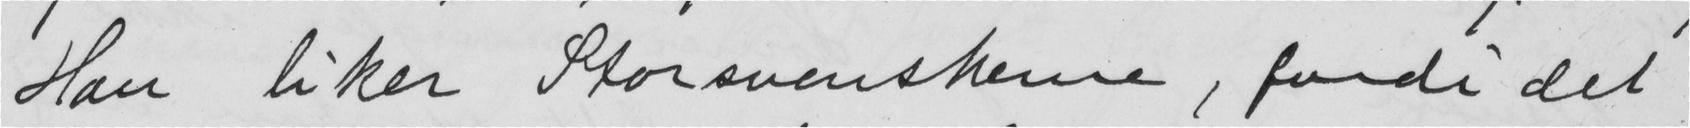Han liker Storsvenskerne, forde det
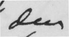den
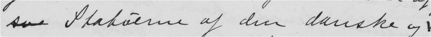Stabuerne af den danske og
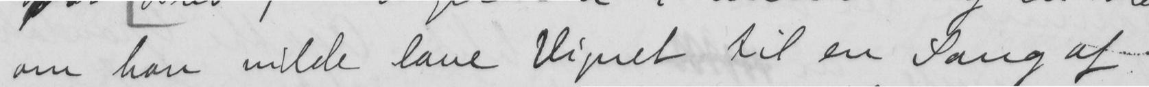om han vilde lave Vignet til en Sang af
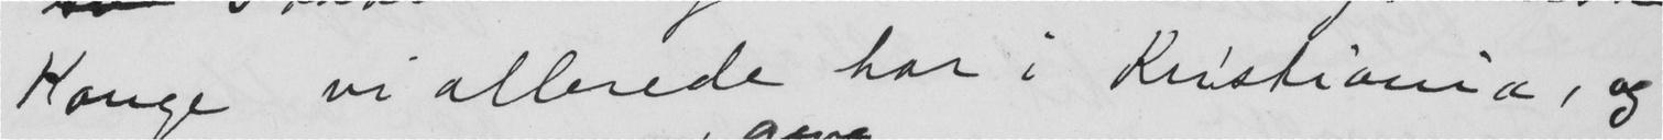Konge vi allerede har i Kristiania, og
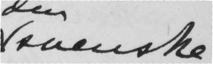Svenske
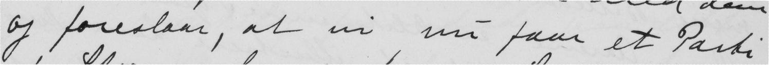og foreslaar, at vi nu faar et Parti
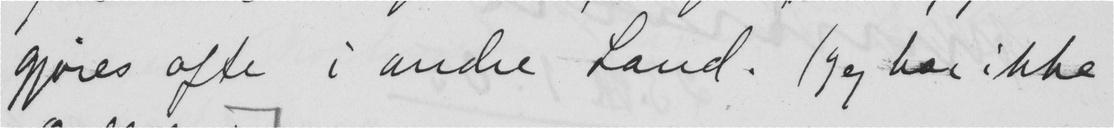gjøres ofte i andre Land. (Jeg har ikke
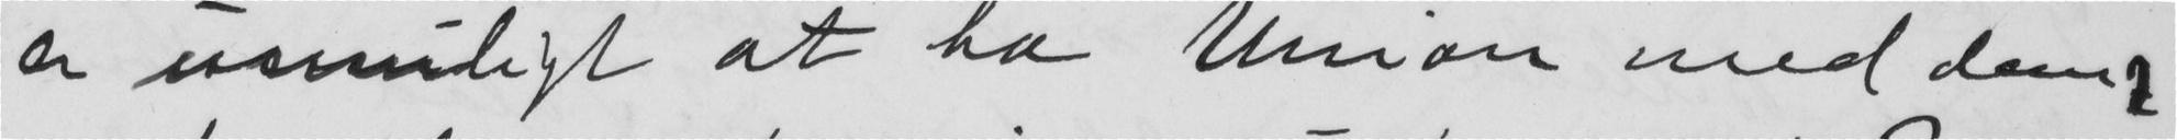er umuligt at ha Union med dem
